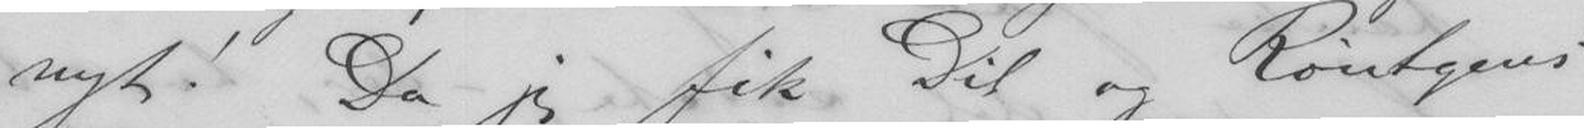nyt! Da jeg fik Dit og Röntgens
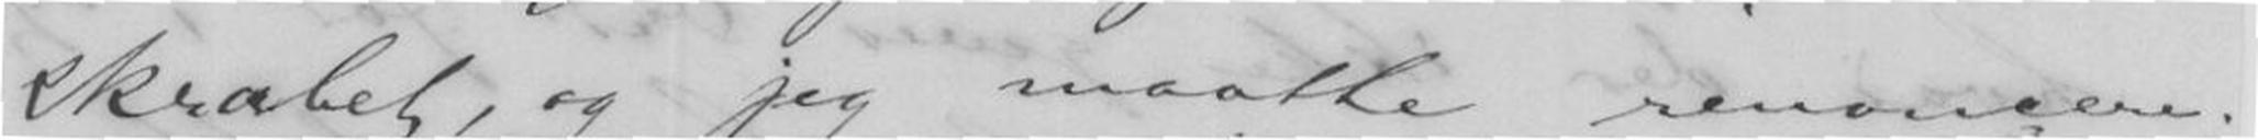skrabet, og jeg maatte renoncere.
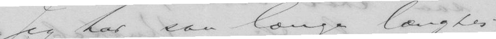Jeg har saa længe længtes
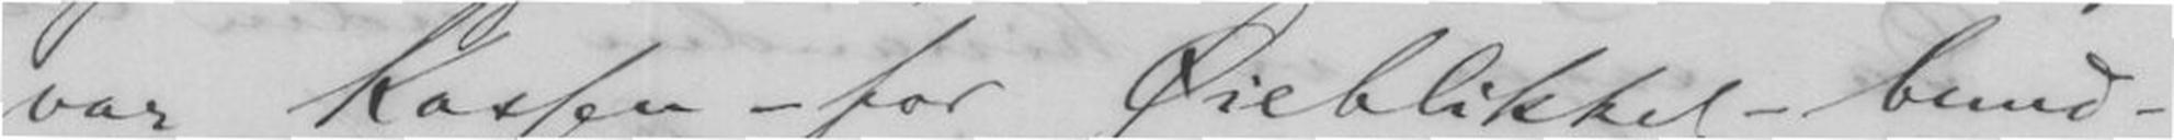var Kassen - for Øieblikket - bund-
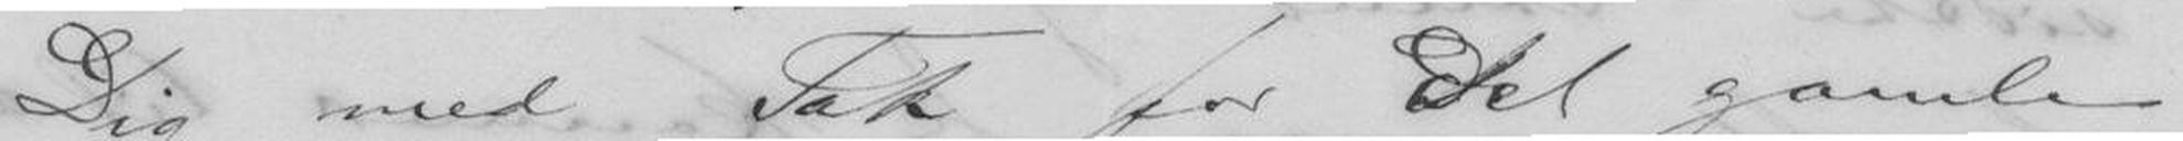Dig med Tak for det gamle
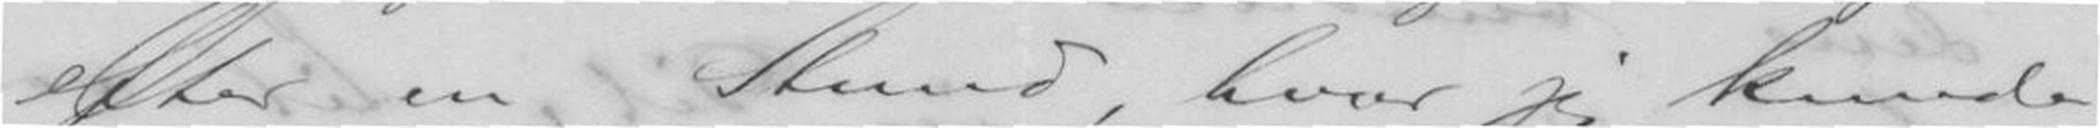efter en Stund, hvor jeg kunde
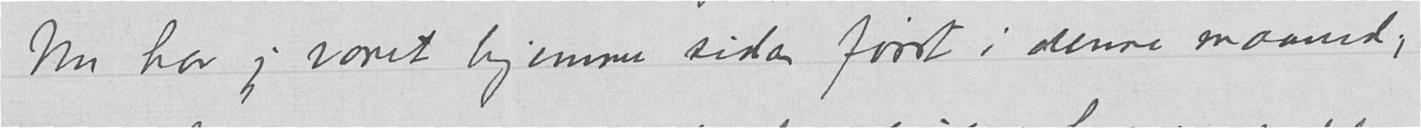Nu har jeg været hjemme siden først i denne maaned;
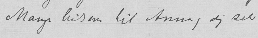Mange hilsener til Anna og dig selv
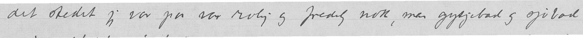det stedet jeg var paa var rolig og fredelig nok, men gytjebad og sjøbad
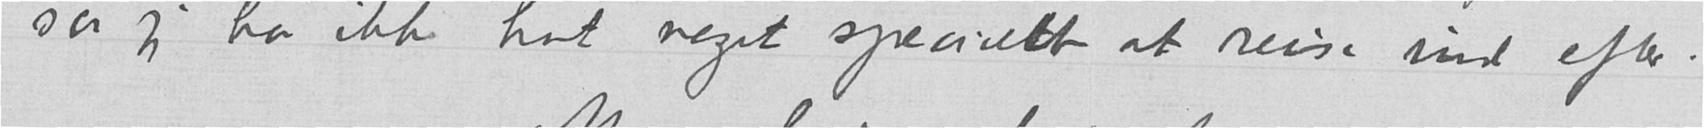saa jeg har ikke hat noget specielt at reise ind efter.
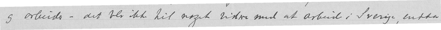og arbeider – det blev ikke til noget videre med at arbeide i Sverige, endda
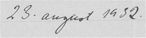23. august 1932.
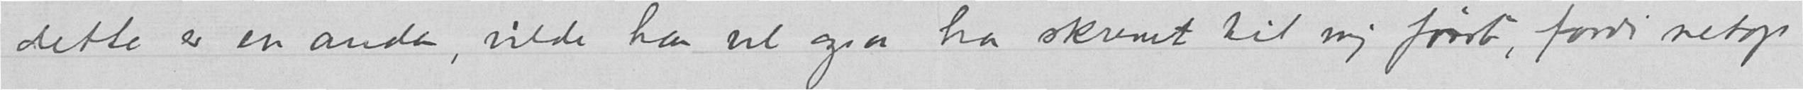dette er en anden, vilde han vel ogsaa ha skrevet til mig først, fordi netop
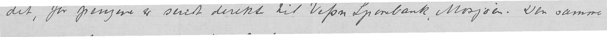det, for pengene er sendt direkte til Vefsn Sparebank, Mosjøen. Den samme
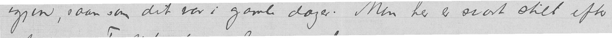igjen, saan som det var i gamle dager. Men her er svært stilt efter
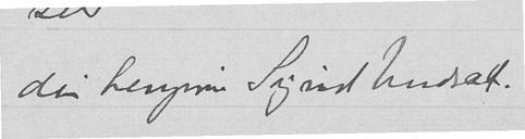din hengivne Sigrid Undset.
